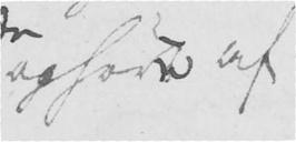ophøre af
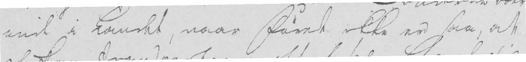inde i Landet, naar føret ikke er saa, at
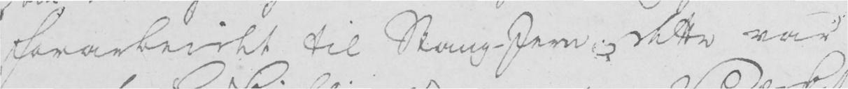forarbeidet til Stang-Jern; dette var
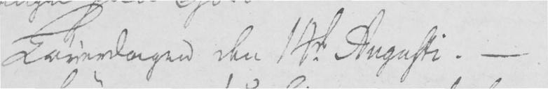Løverdagen den 14de Augusti. -
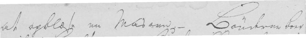at opblæse en Masovn; - Bønderne boer
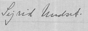Sigrid Undset.
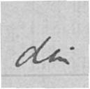din
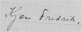Kjære Fredrik,
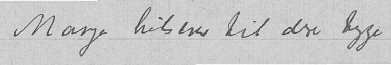Mange hilsener til dere begge
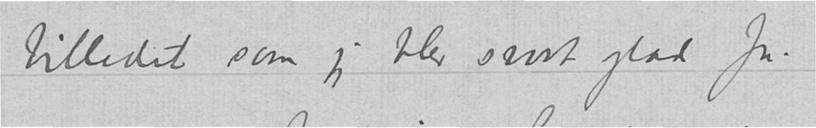billedet som jeg blev svært glad for.
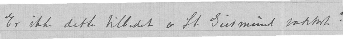Er ikke dette billedet av St. Gudmund vakkert?
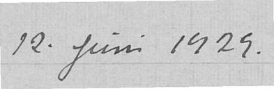12.juni 1929.
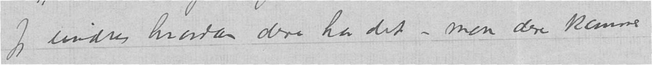Jeg undres hvordan dere har det - mon dere kommer
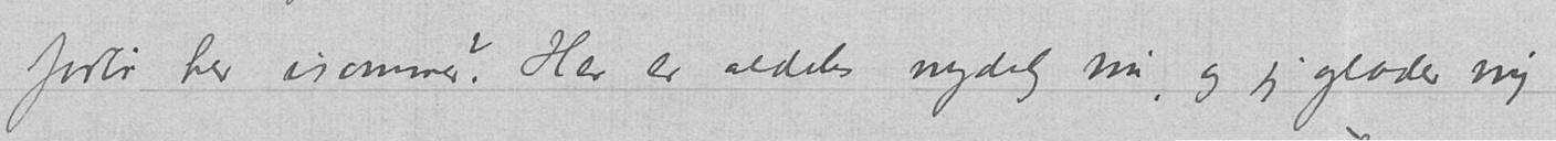forbi her isommer? Her er aldeles nydelig nu, og jeg glæder mig
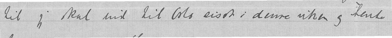til jeg skal ind til Oslo sisst i denne uken og hente
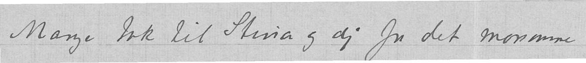Mange tak til Stina og dig for det morsomme
